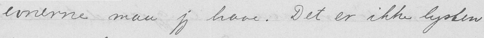evnerne maa jeg have. Det er ikke lysten
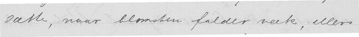sætte, naar blomsten falder væk, ellers
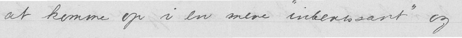at komme op i en mere "interessant" og
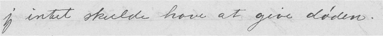jeg intet skulde have at give døden.
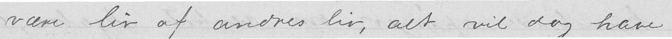være liv af andres liv, alt vil dog have
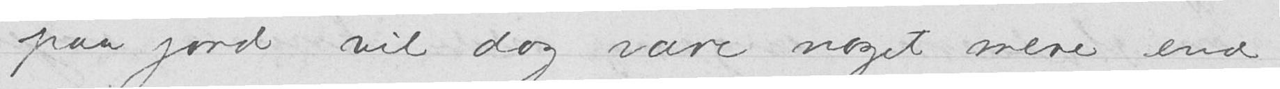paa jord vil dog være noget mere end
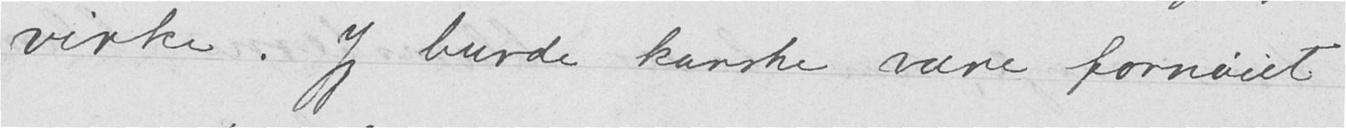virke. Jeg burde kanske være fornøiet
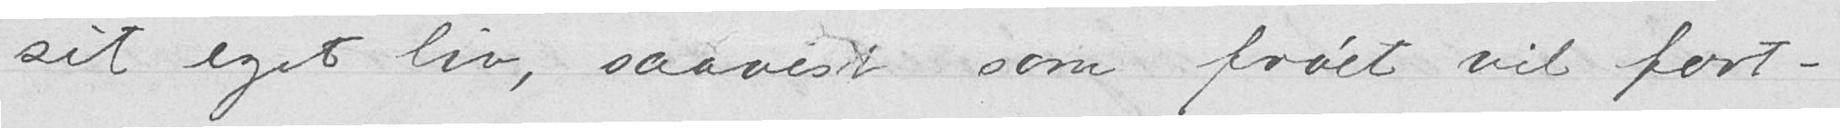sit eget liv, saavist som frøet vil fort-
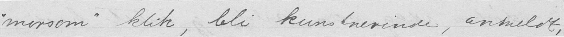"morsom" klik, bli kunstnerinde, anmeldt,
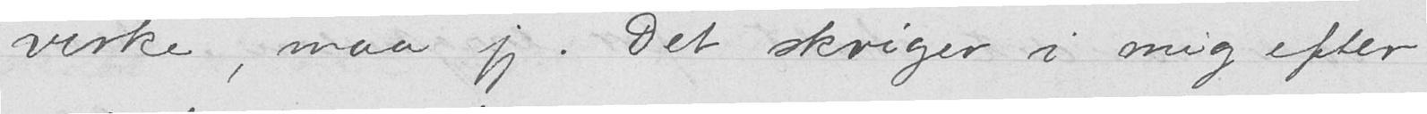virke, maa jeg. Det skriger i mig efter
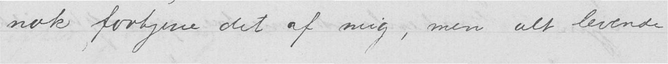nok fortjene det af mig, men alt levende
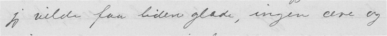jeg vilde faa liden glæde, ingen ære og
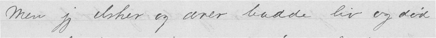Men jeg elsker og ærer baade liv og død
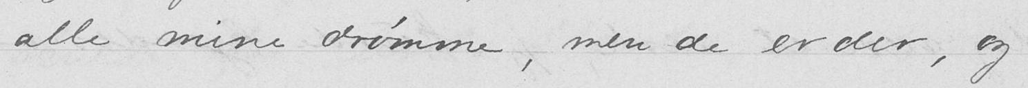alle mine drømme, men de er der, og
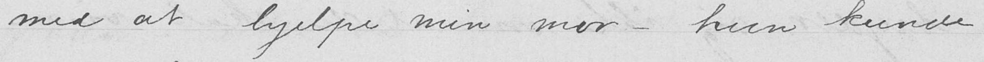med at hjelpe min mor - hun kunde
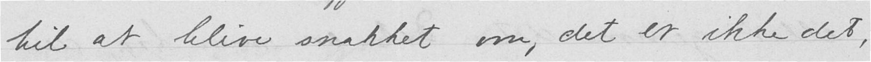til at blive snakket om, det er ikke det,
